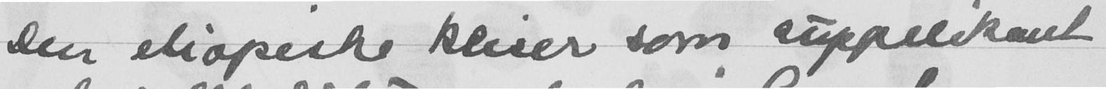Den etiopiske keiser som supplikant
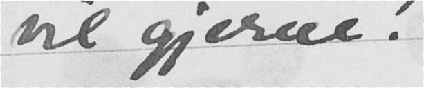vil gjerne!
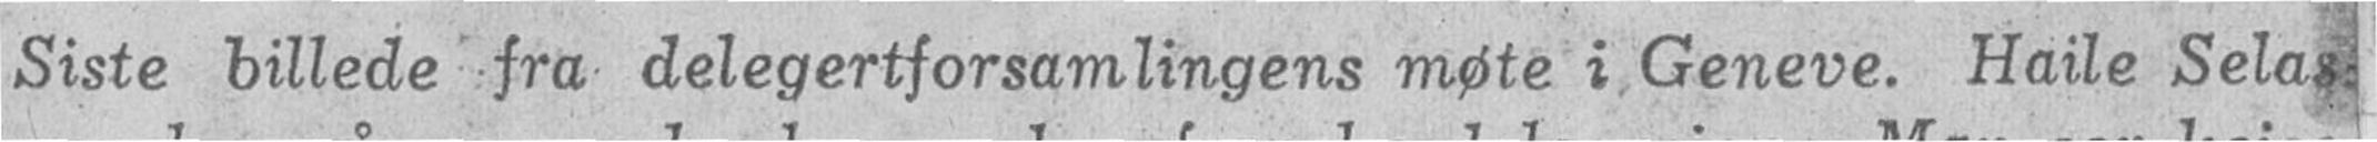Siste billede fra delegertforsamlingens møte i Geneve. Haile Selas-
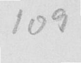109
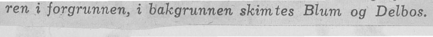ren i forgrunnen, i bakgrunnen skimtes Blum og Delbos.
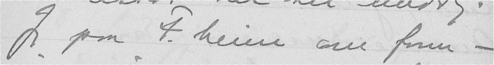Jeg paa F. heim om form -
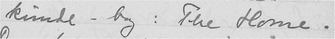kvinde-bog: The Home.
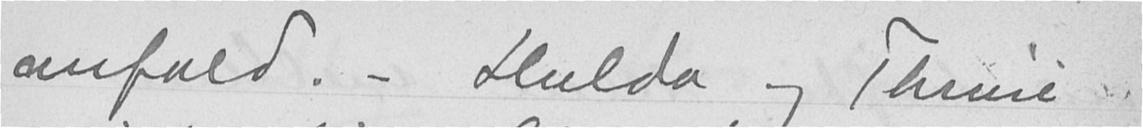anfald. - Hulda og Thrine
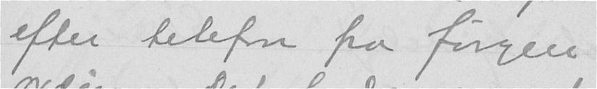efter telefon fra Jørgen
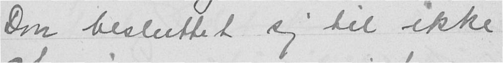Don besluttet sig til ikke
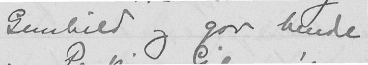Gunhild og gav hende
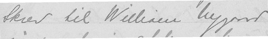Skrev til William Nygaard
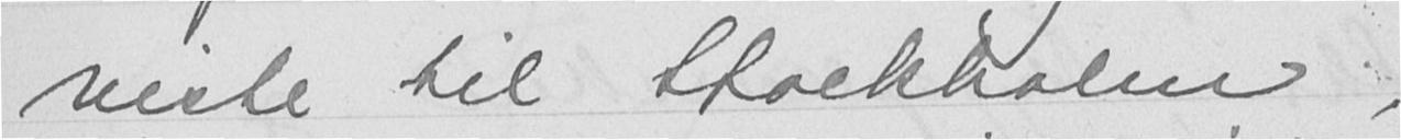reiste til Stockholm,
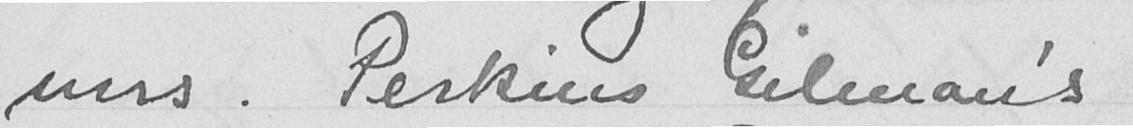mrs. Perkins Gilman's
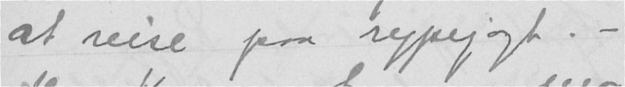at reise paa rypejagt. -
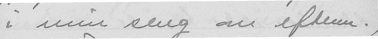i min seng om efterm.,
